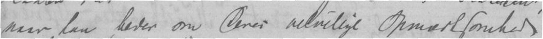naar han om Deres velvilige Opmærksomhed
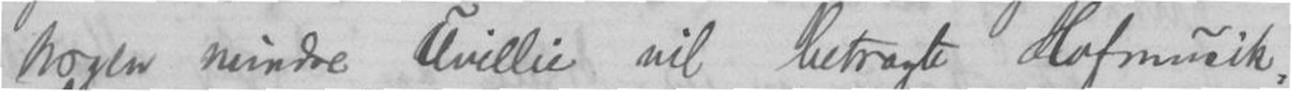mindre Uvillie vil betragte Hofmusik-
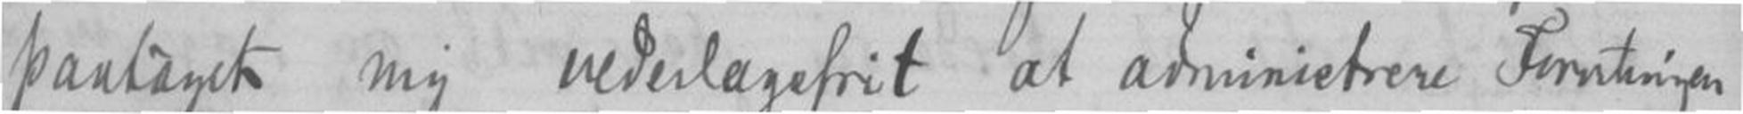paatænkt mig vederlagefrit at adminictrere Forretningen
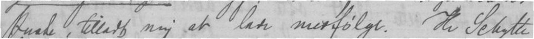, tilladt mig at lade medfølge. Hr Schytte
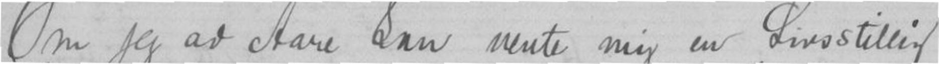Om jeg ad Aare kan vente mig en Livsstilling
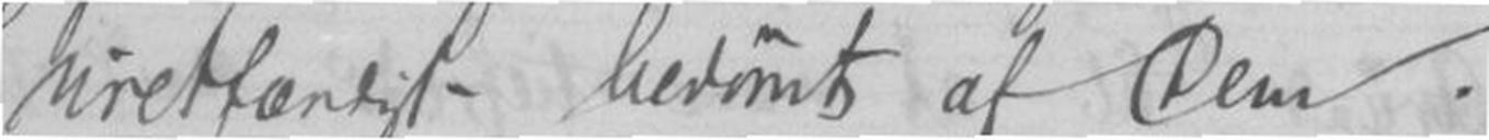Uretfærdigt bedømts af Dem.
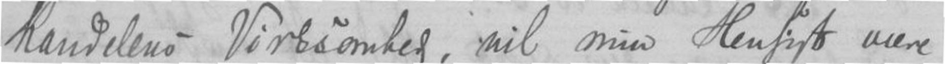handelens Virksomhed, vil min hensikt være
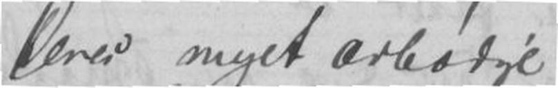Deres meget ærbødige
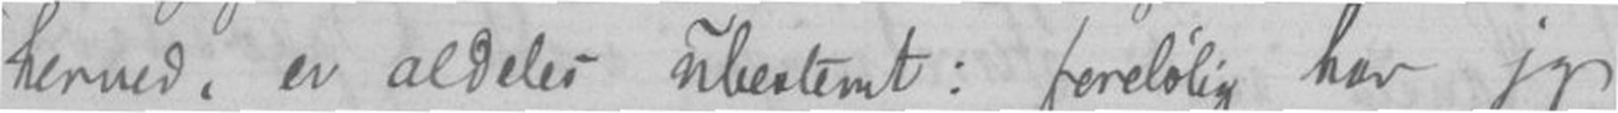herved, er aldeles ubestemt: foreløbig har jeg
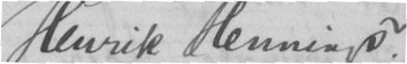Henrik Hennings
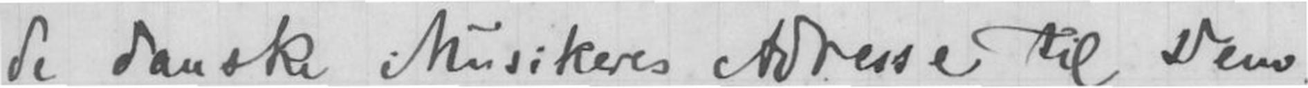de danske Musikeres Adresse til Dem
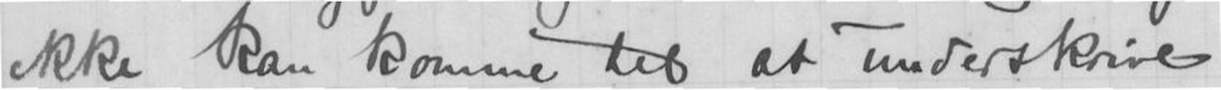ikke kan komme til at underskrive
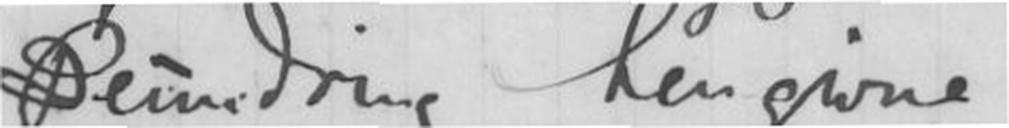i Beundring hengivne
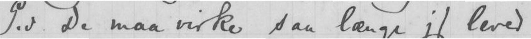Giv De maa virke saa længe jeg lever.
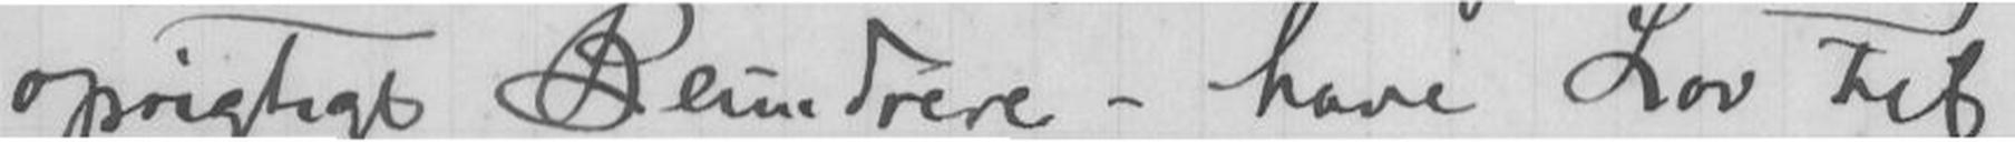oprigtigt Beundrere - have Lov til
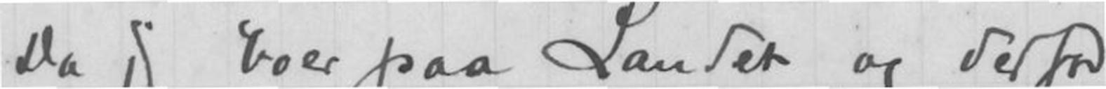Da jeg boer paa Landet og derfor
